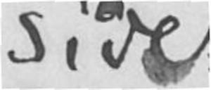side
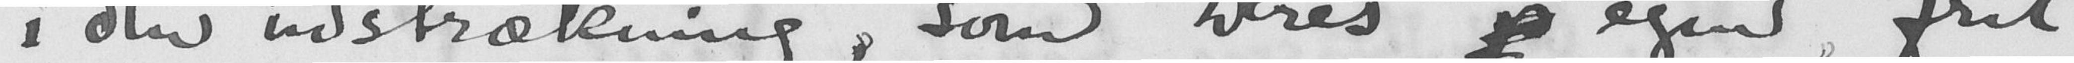i den udstrækning, som Deres p egen, frit
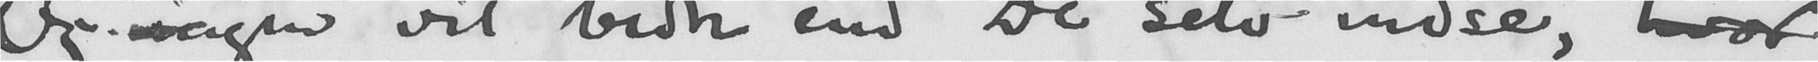Og ingen vil bedre end De selv indse, hvor
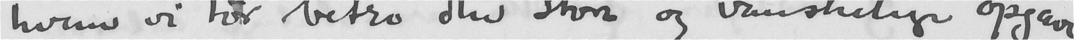hvem vi tør betro den store og vanskelige opgave.
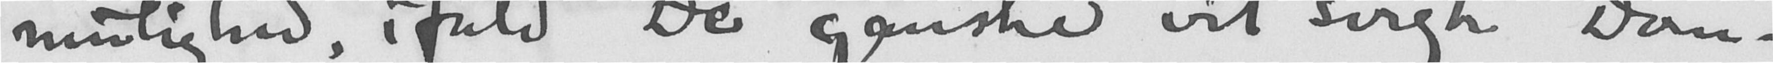mulighed, ifald De ganske vil svigte Dom-
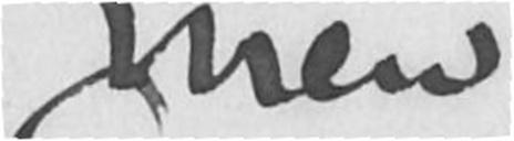Men
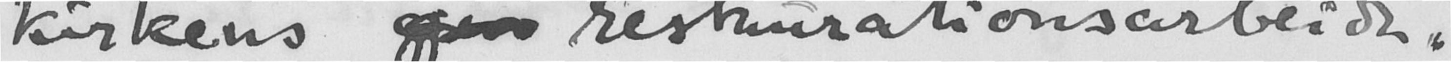kirkens gjen restaurationsarbeide.
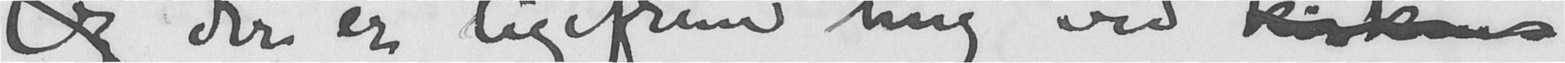Og der er ligefrem ting ved kirkens
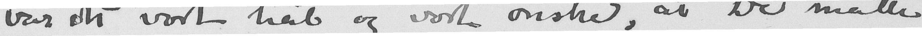var det vort håb og vort ønske, at De måtte
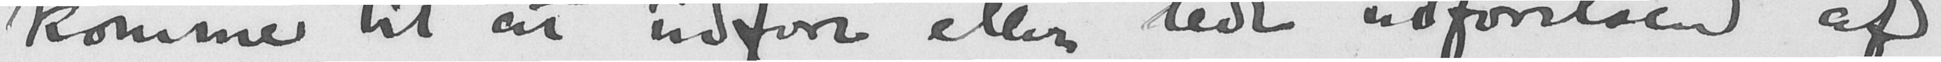komme til at udføre eller lede udførelsen af
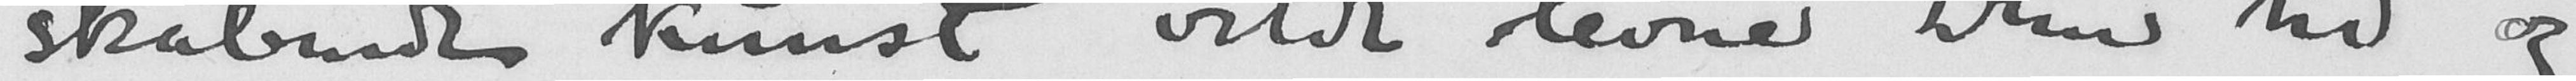skabende kunst vilde levne Dem tid og
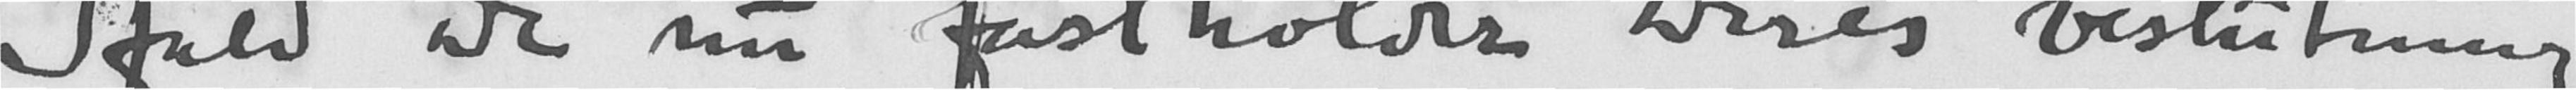Ifald De nu fastholder Deres beslutning
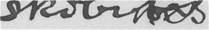skibes
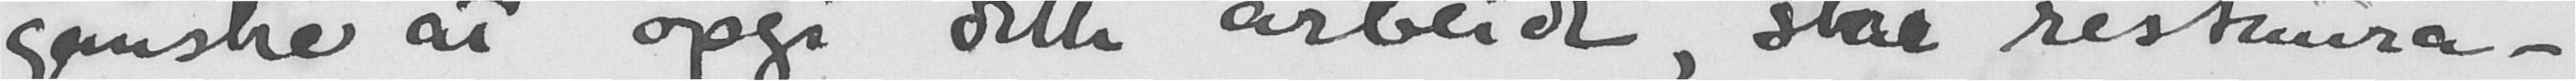ganske at opgi dette arbeide, står restaura-
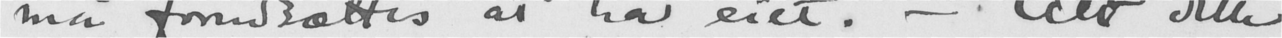må forudsættes at ha eiet. - Alt dette
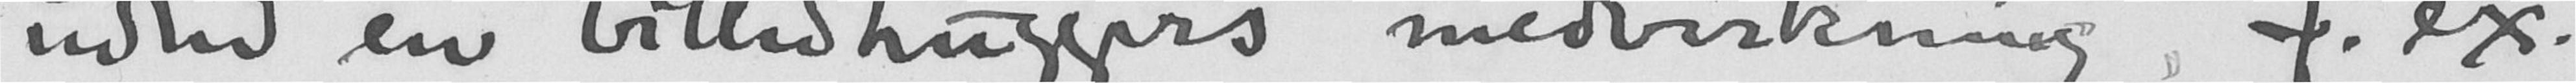uden en billedhuggers medvirkning, f.ex.
